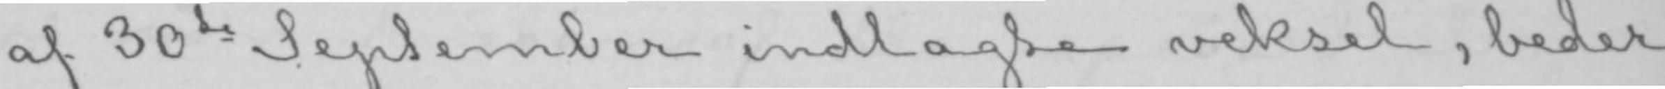af 30te September indlagte veksel, beder
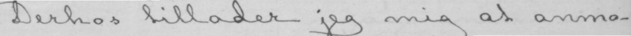Derhos tillader jeg mig at anmo-
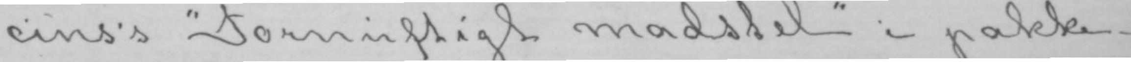cius's «Fornuftigt madstel» i pakke-
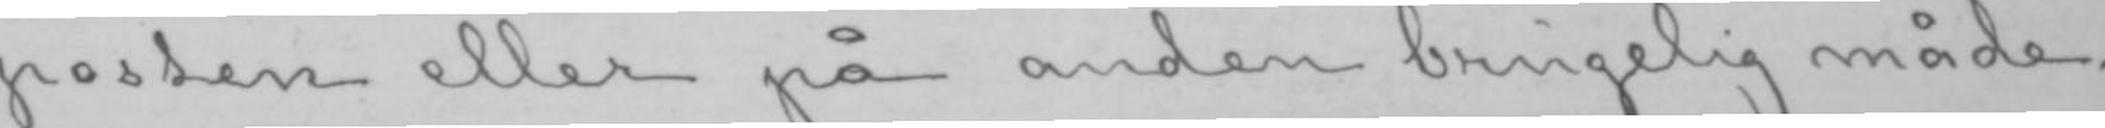posten eller på anden brugelig måde.
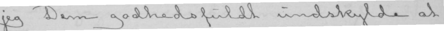jeg Dem godhedsfuldt undskylde at
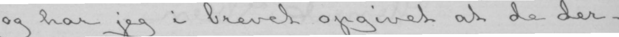og har jeg i brevet opgivet at de der-
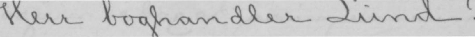Herr boghandler Lund!
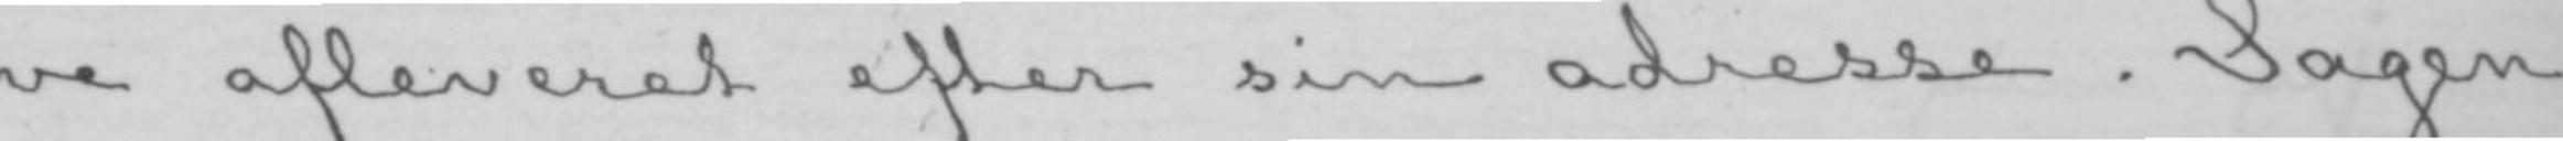ve afleveret efter sin adresse. Sagen
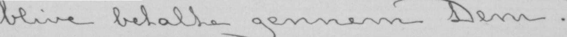blive betalte gennem Dem.
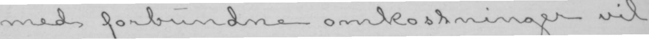med forbundne omkostninger vil
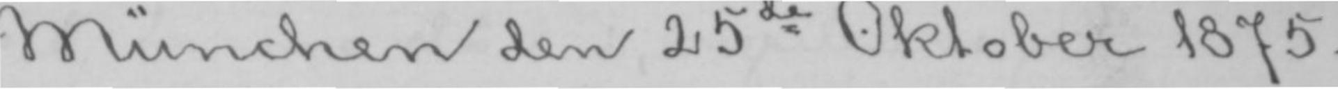München den 25de Oktober 1875.
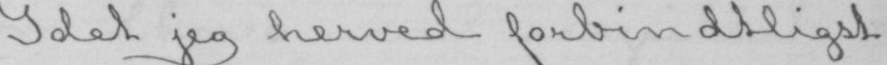Idet jeg herved forbindtligst
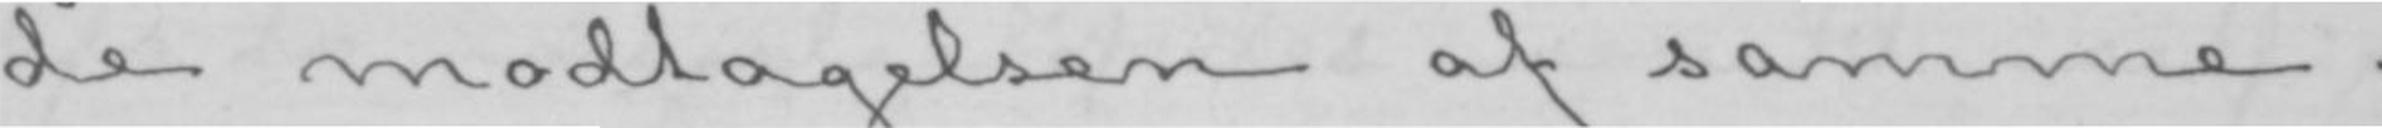de modtagelsen af samme.
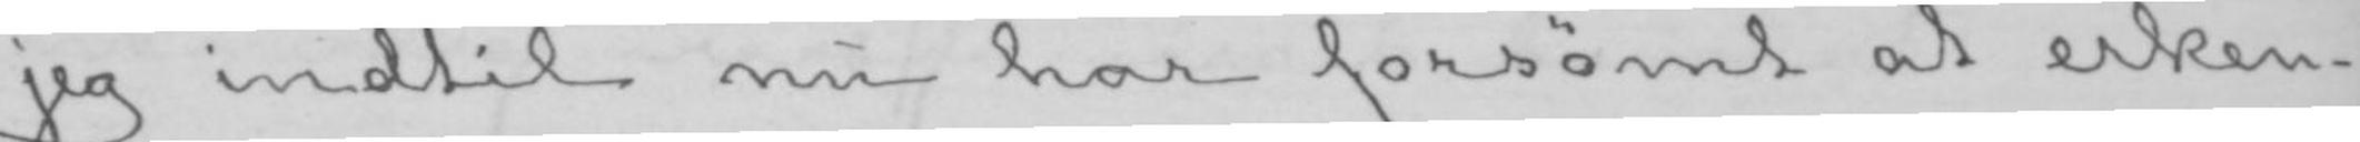jeg indtil nu har forsømt at erken-
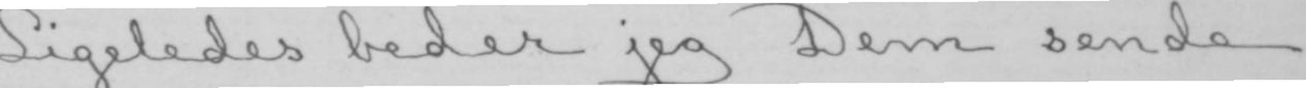Ligeledes beder jeg Dem sende
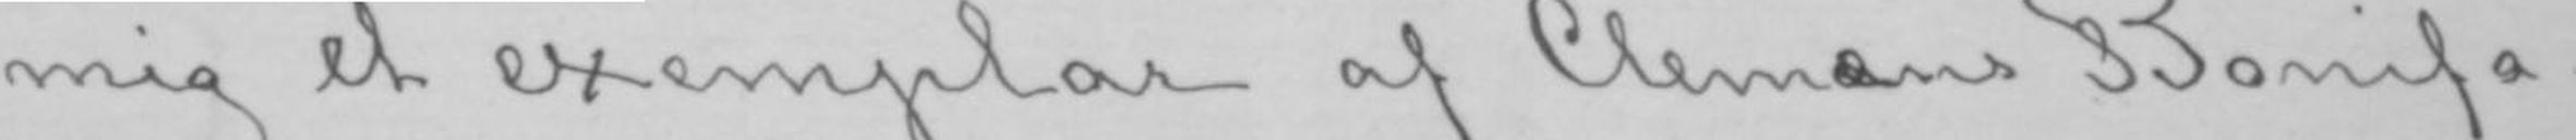mig et exemplar af Clemaens Bonifa-
